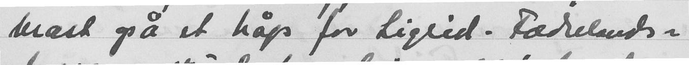brast også et håp for Sigrid. Fædrelands-
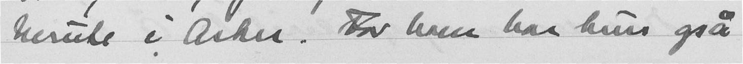herute i Asker. For ham har hun også
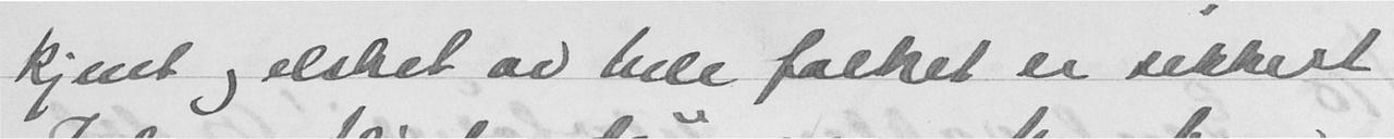kjent og elsket av hele folket er sikkert
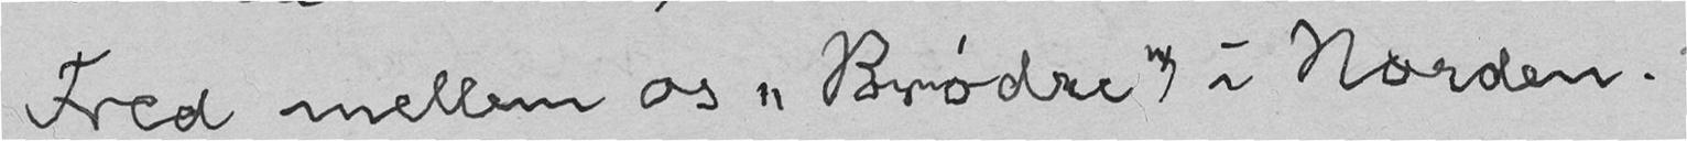Fred mellem os "Brødre" i Norden.
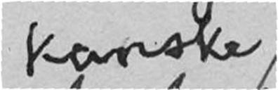kanske
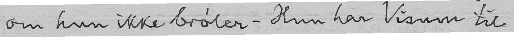om hun ikke brøler. Hun har Visum til
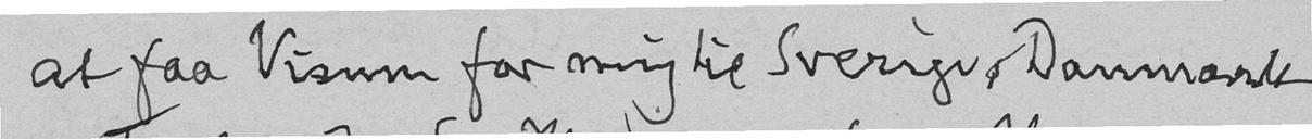at faa Visum for mig til Sverige, Danmark
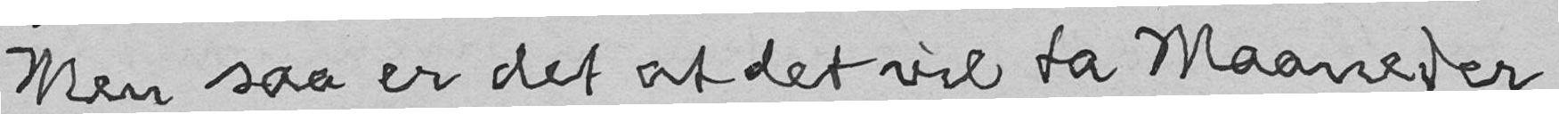Men saa er det at det vil ta Maaneder
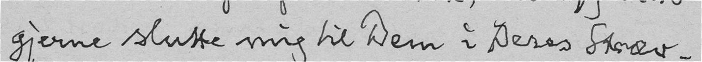gjerne slutte mig til Dem i Deres Stræv.
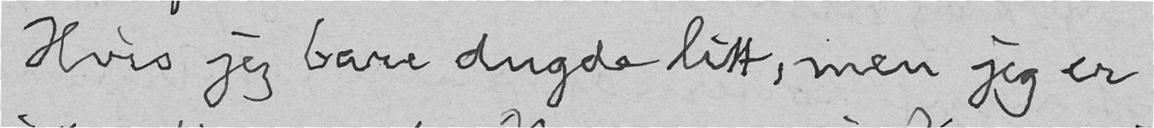Hvis jeg bare dugde litt, men jeg er
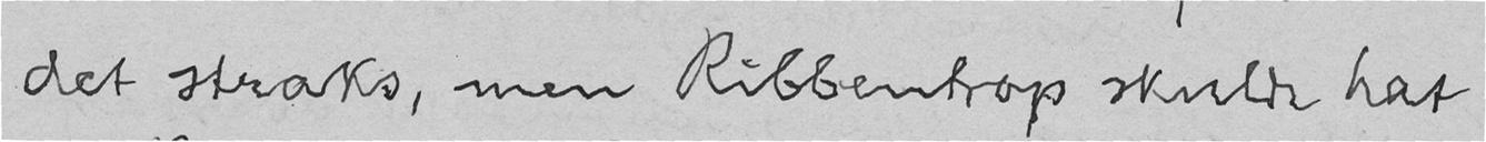det straks, men Ribbentrop skulde hat
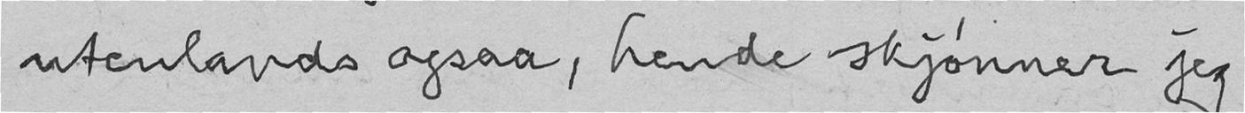utenlands ogsaa, hende skjønner jeg
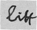litt
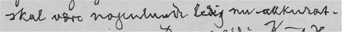skal være nogenlunde ledig nu akkurat.
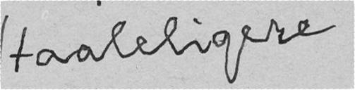taaleligere
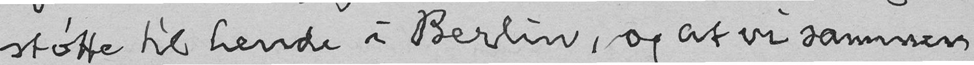støtte til hende i Berlin, og at vi sammen
